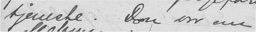tjeneste. Don var om
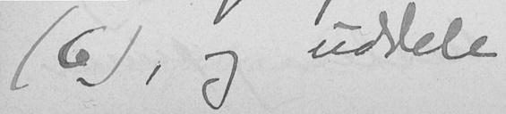(6), og uddele
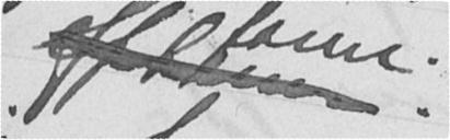efterm.
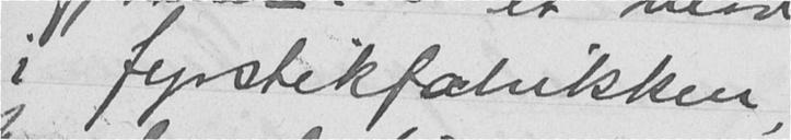i fyrstikfabrikken,
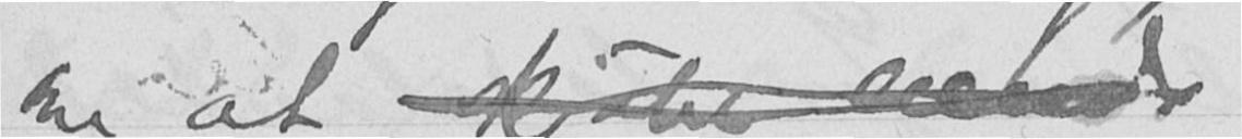om at kjøbe eiend
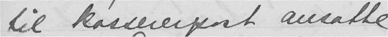til kassererpost ansatte
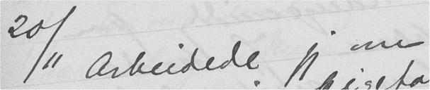20/11 Arbeidede jeg om
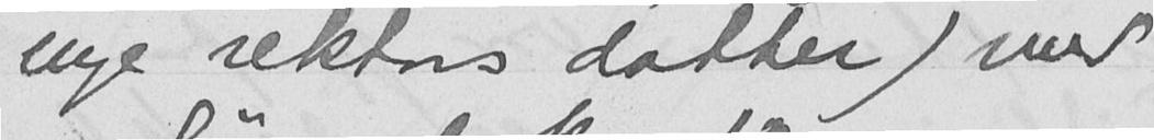nye rektors datter) med
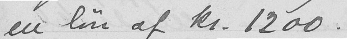en løn af kr. 1200.
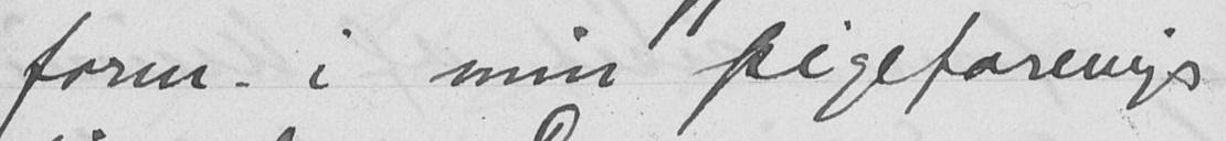form. i min pigeforenings
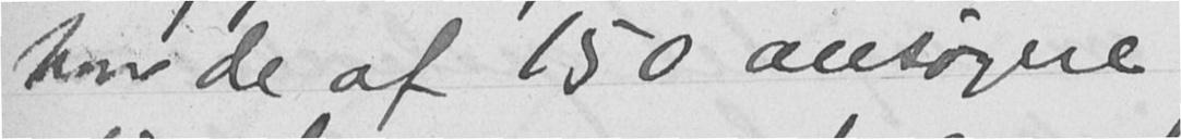hvor de af 150 ansøgere
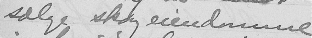sælge skogeiendomme
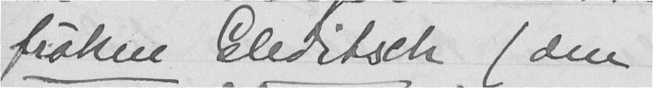frøken Gleditsch (den
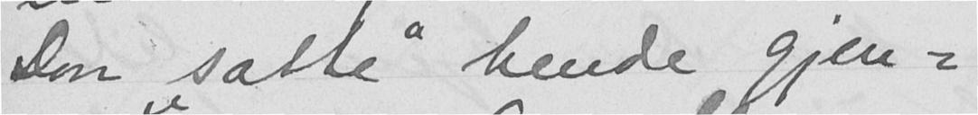Don "satte" hende gjen-
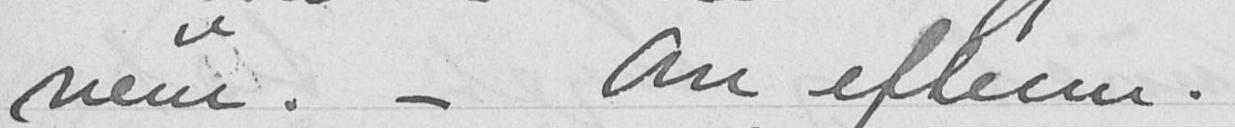mem. - Om efterm.
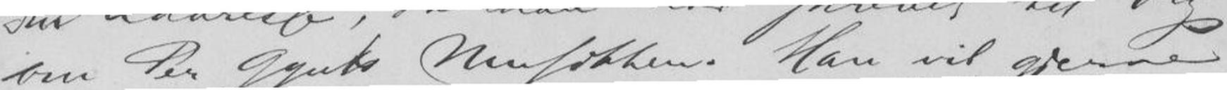om Per Gynt Musikken. Han vil gjerne
